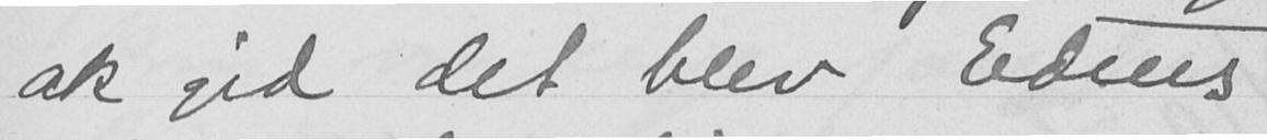ak gid det blev Edens
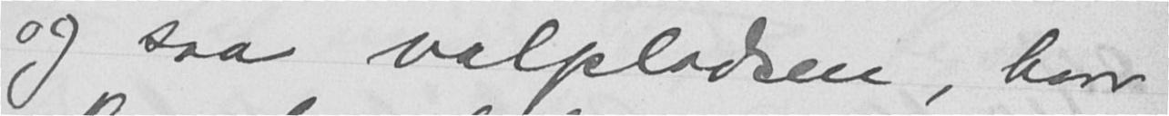og saa valpladsen, hvor
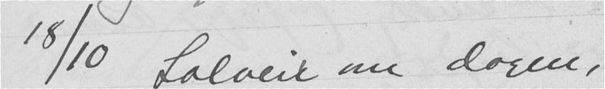18/10 Solveir om dagen,
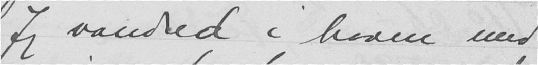Jeg vandred i haven med
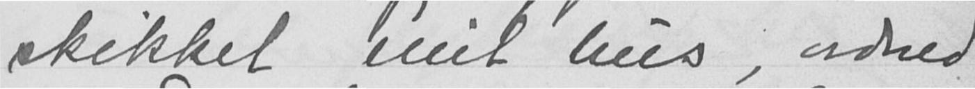skikket mit hus, ordned
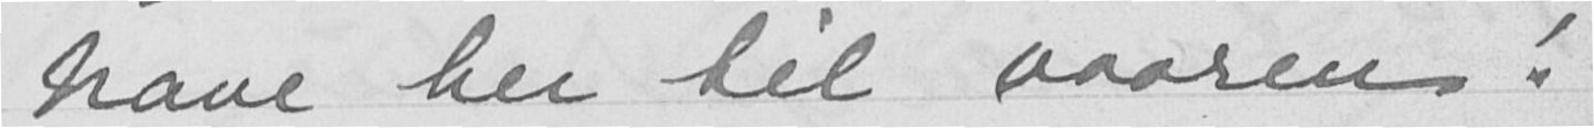have her til vaaren!
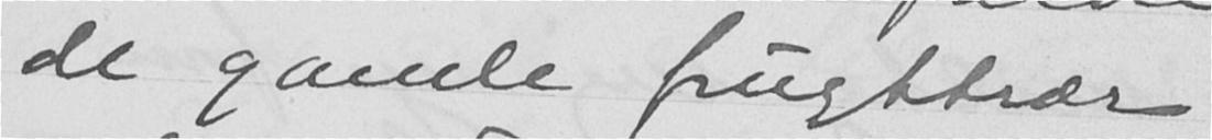de gamle frugttrær
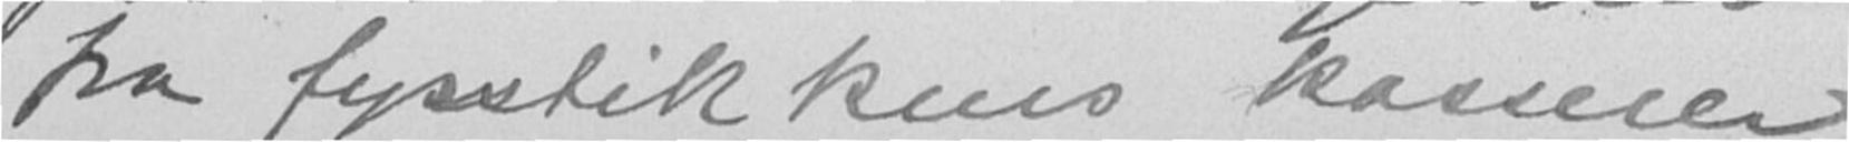fra fyrstikkens kasserer
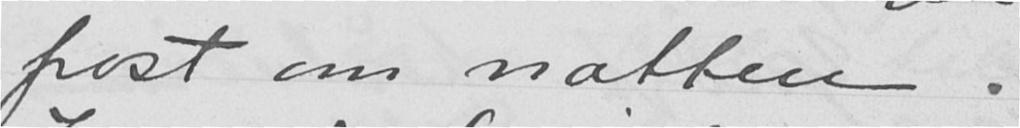frost om natten.
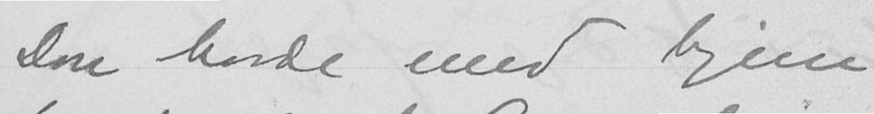Don havde med hjem
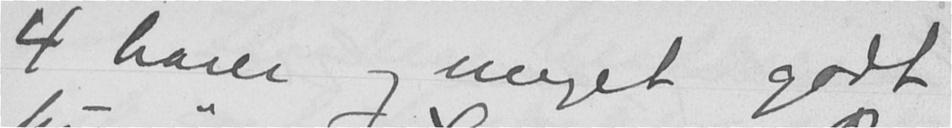4 harer og meget godt
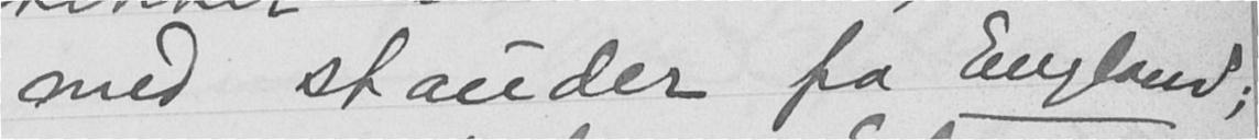med stauder fra England;
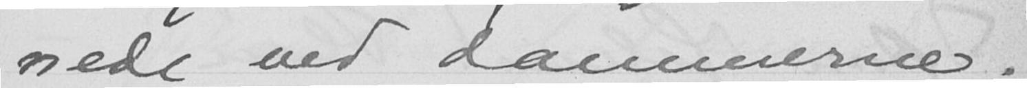nede ved dammerne.
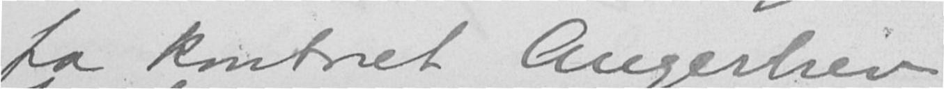fra kontoret Angerbrev
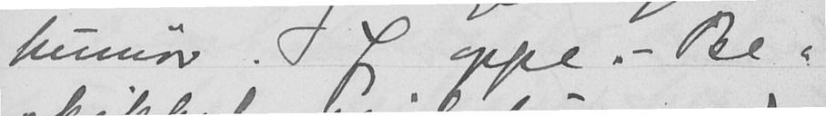humør. Jeg oppe. - Be-
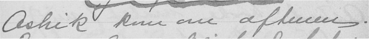Astrik kom om aftenen.
